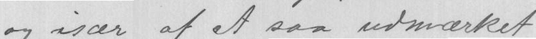og især af et saa udmærket
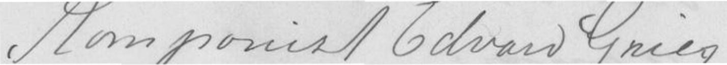Komponist Edvard Grieg
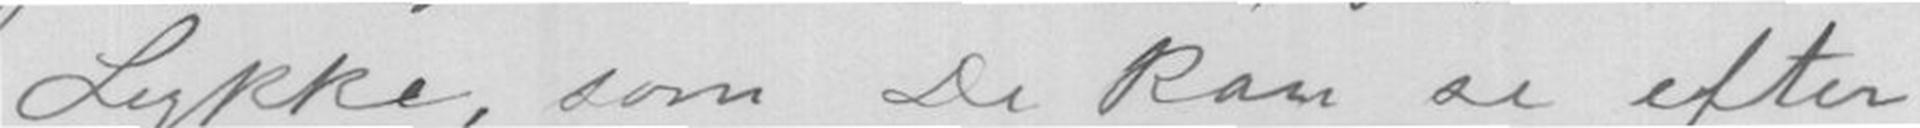Lykke, som De kan se efter
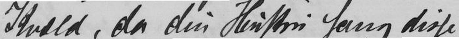Kvæld, da din Hustru sang disse
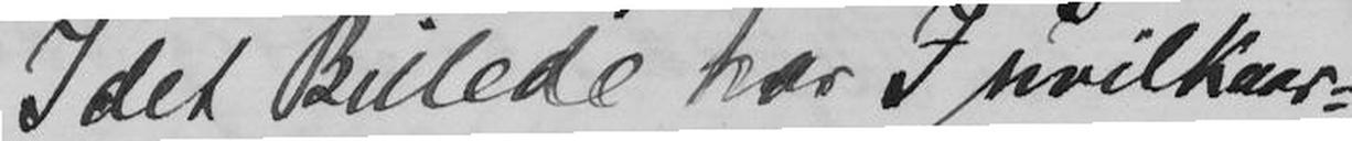I det Billede har I uvilkaar-
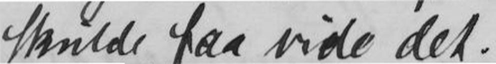skulde faa vide det.
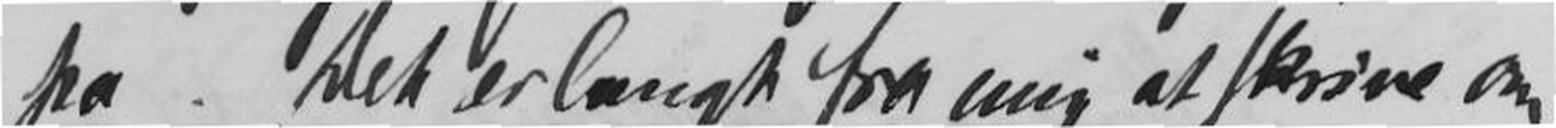pa. Det er langt fra mig at skrive om
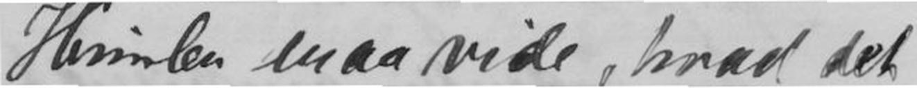Himlen maa vide, hvad det
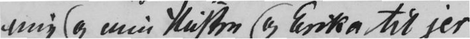mig og min Hustru og Erika til jer
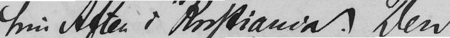hin Aften i Kristiania. Den
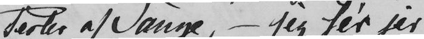Perler af Sange, - jeg ser jer
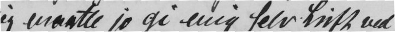jeg maatte jo gi mig selv Luft ved
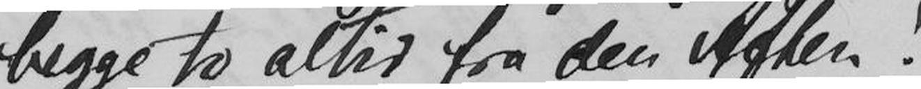begge to altid fra den Aften!
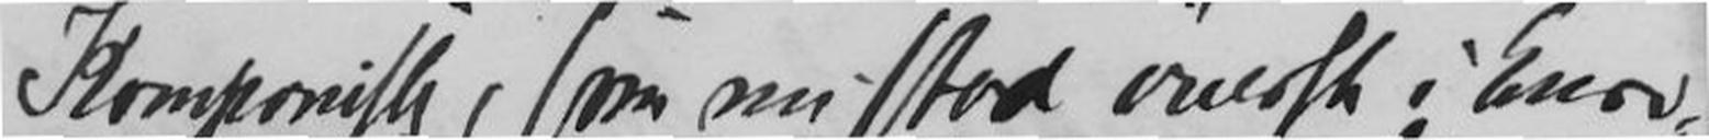Komponist, som nu stod øverst i Euro-
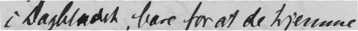i Dagbladet, bare for at de hjemme
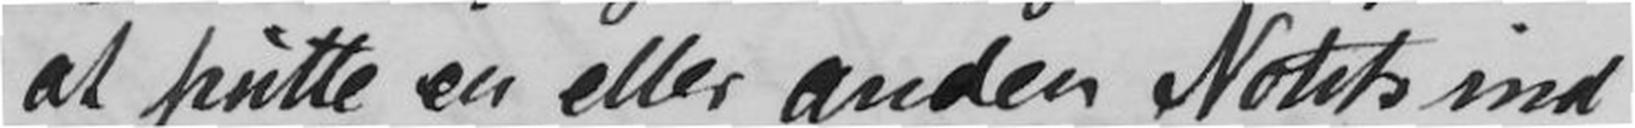at putte en eller andet Notits ind
